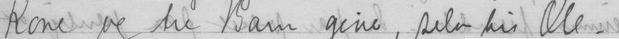Kone og tre Barn give, selv til Ole.
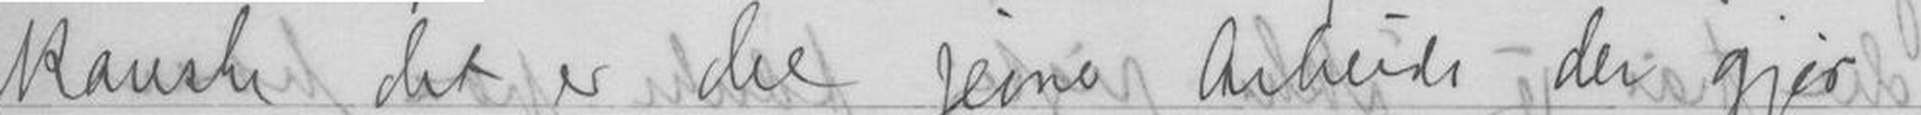kanske det er det jevne Arbeide der gjør
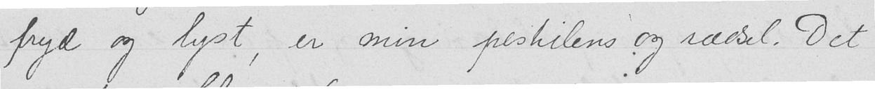fryd og lyst, er min pestilens og rædsel. Det
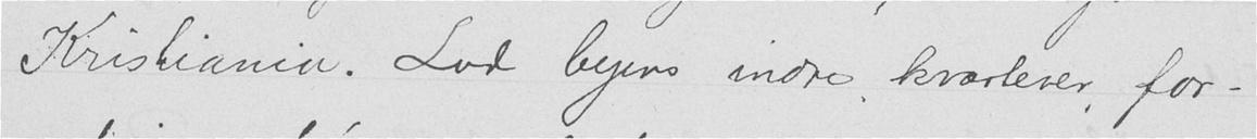Kristiania. Lad byens indre kvarterer, for-
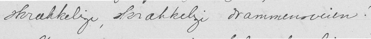skrækkelige, skrækkelige Drammensveien!
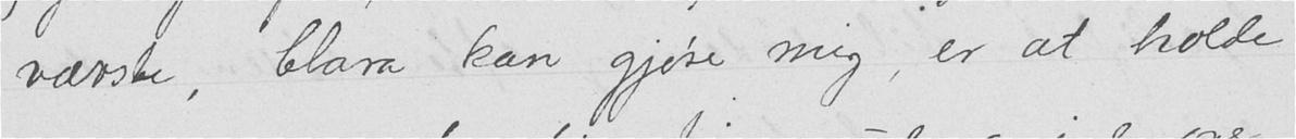værste, Clara kan gjøre mig, er at holde
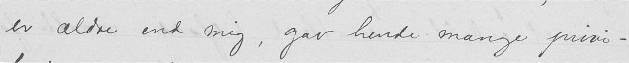er ældre end mig, gav hende mange privi-
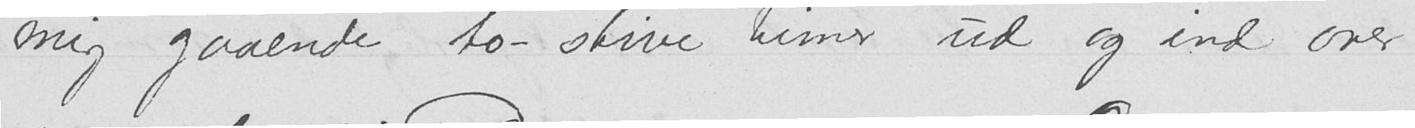mig gaaende to stive timer ud og ind over
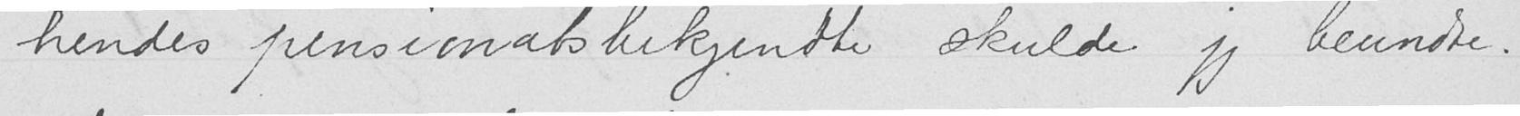hendes pensionatsbekjendte skulde jeg beundre.
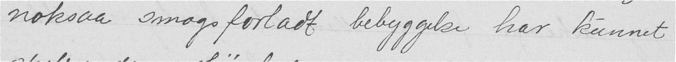noksaa smagsforladt bebyggelse har kunnet
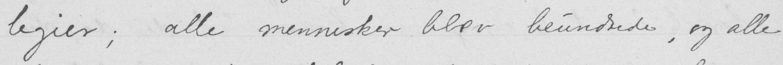legier; alle mennesker blev beundrede og alle
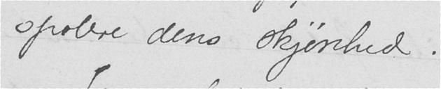spolere dens skjønhed.
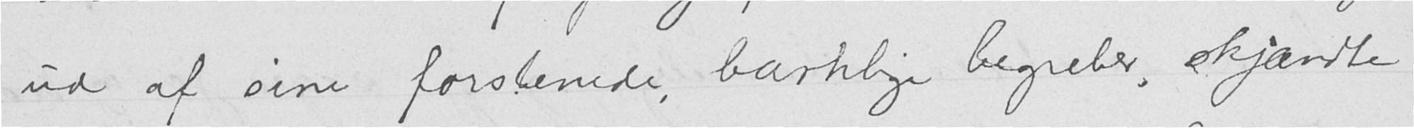ud af sine forstenede, barnlige begreber, skjændte
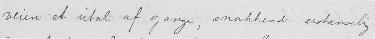veien et utal af gange, snakkende ustanselig
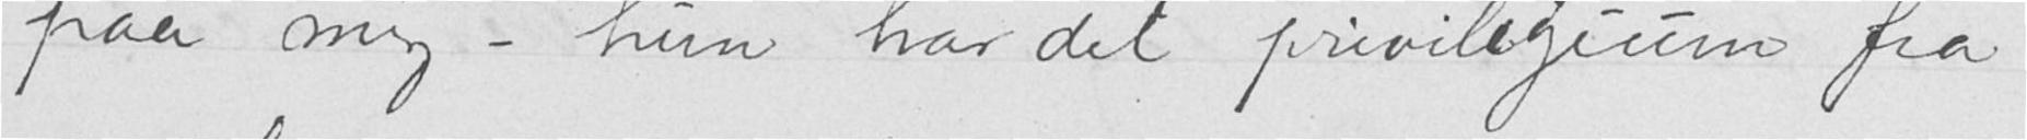paa mig — hun har det privilegium fra
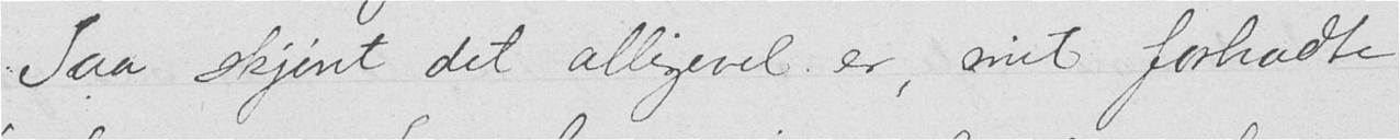Saa skjønt det alligevel er, mit forhadte
